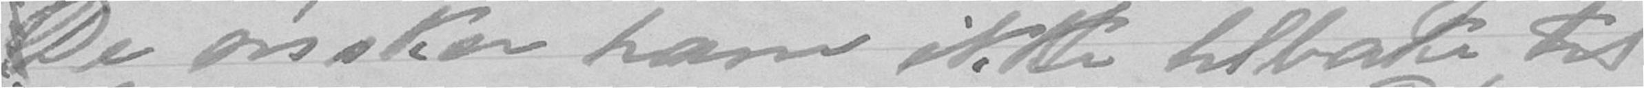De ønsker ham ikke tilbake til
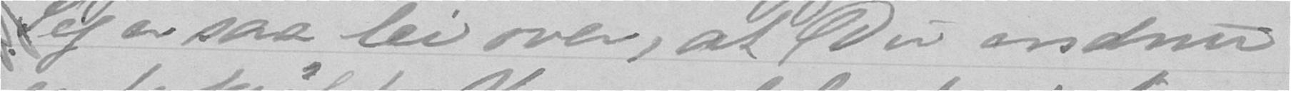Jeg er saa lei over, at Du endnu
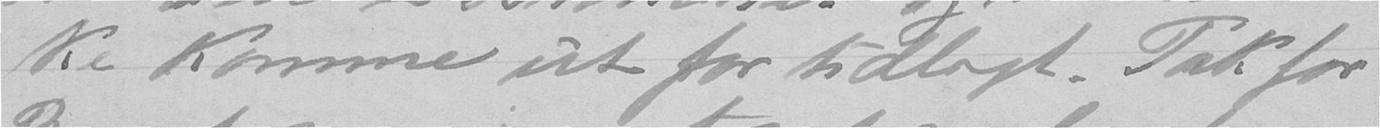ke komme ut for tidligt. Tak for
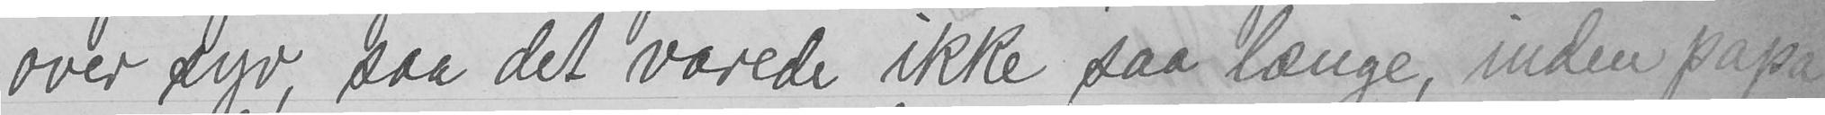over syv, saa det varede ikke saa længe, inden papa
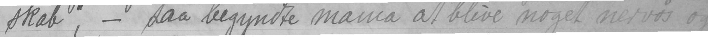skab", - saa begyndte mama at blive noget nervøs og
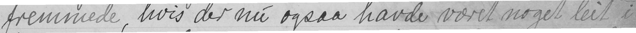fremmede, hvis der nu ogsaa havde været noget leit i
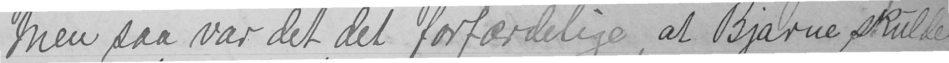Men saa var det det forfærdelige, at Bjarne skulde
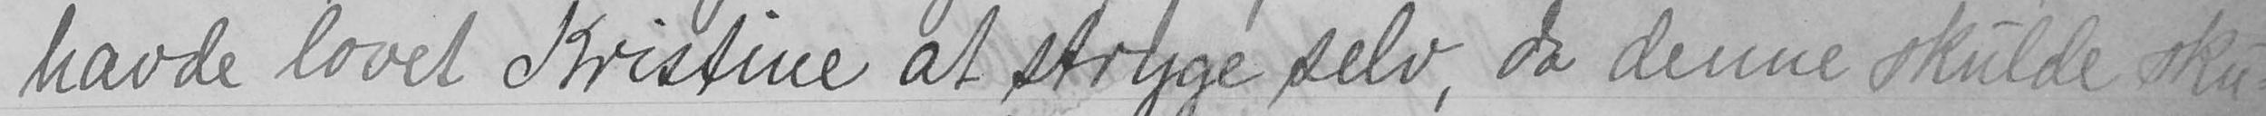havde lovet Kristine at stryge selv, da denne skulde sku-
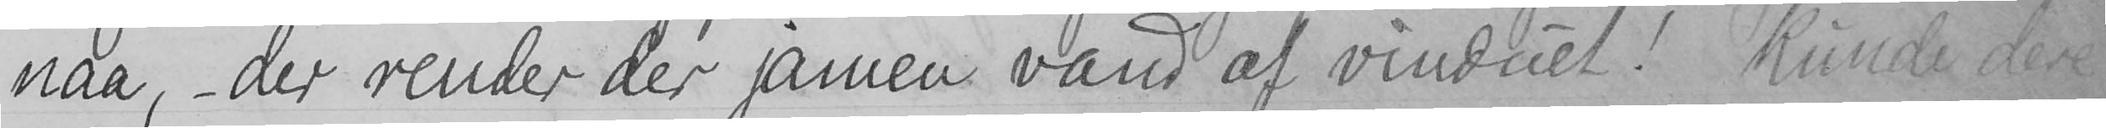naa, - der render der jamen vand af vinduet! kunde dere
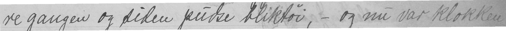re gangen og siden pudse bliktøi, - og nu var klokken
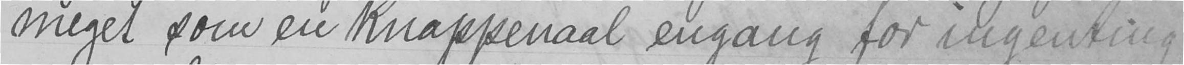meget som en knappenaal engang for ingenting
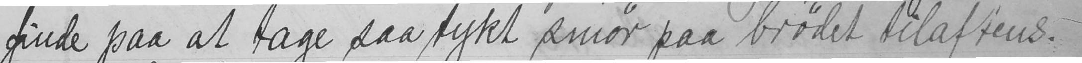finde paa at tage saa tykt smør paa brødet tilaftens.
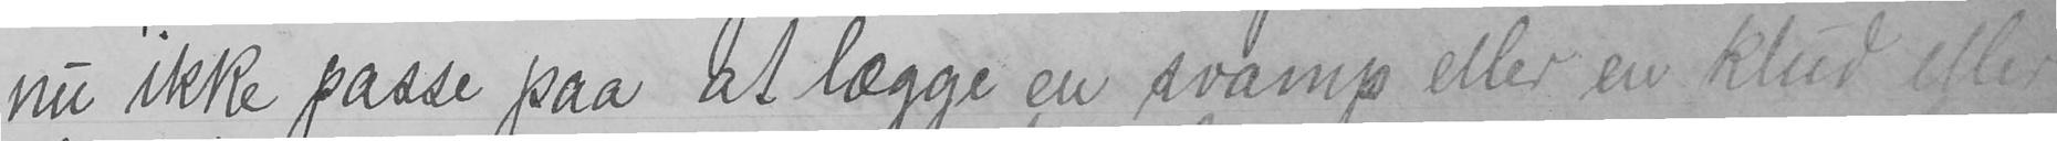nu ikke passe paa at lægge en svamp eller en klud eller
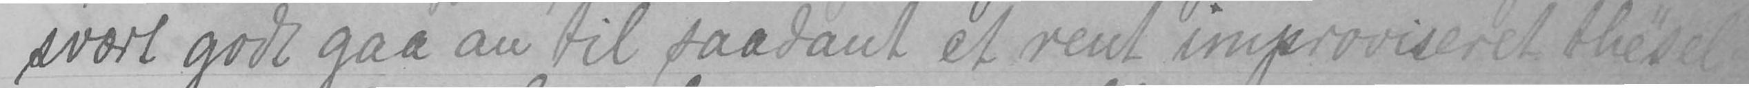svært godt gaa an til saadant et rent improviseret thesel-
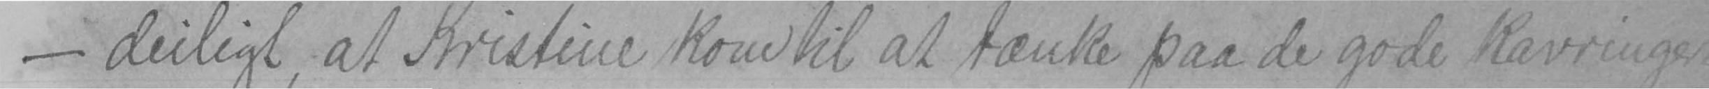- deiligt, at Kristine kom til at tænke paa de gode kavringer-
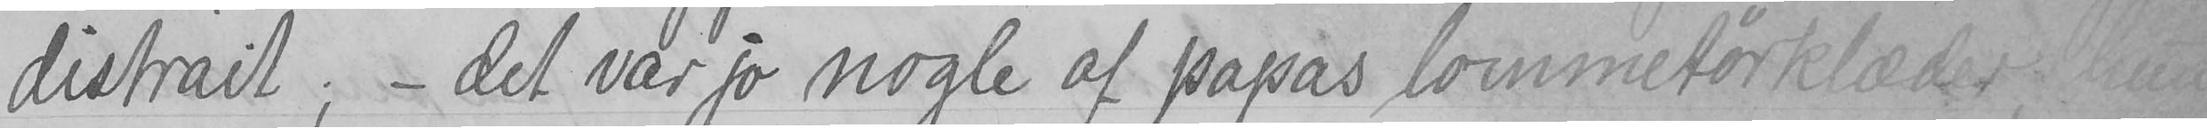distrait; - det var jo nogle af papas lommetørklæder, hun
